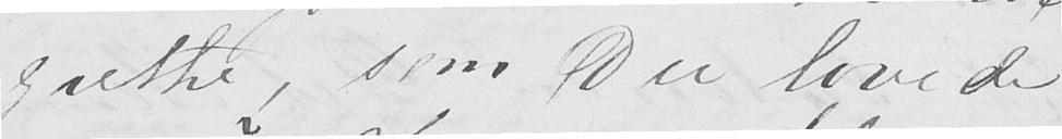grethe, som Du lovede
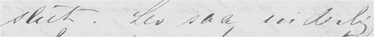slut. Lev saa inderlig
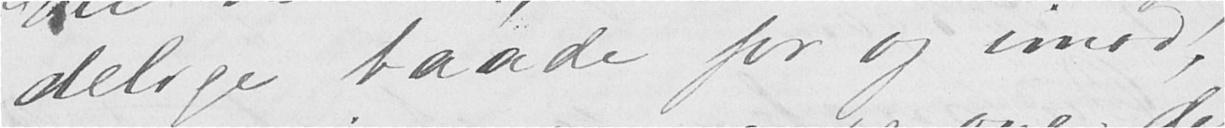delige baade for og imod,
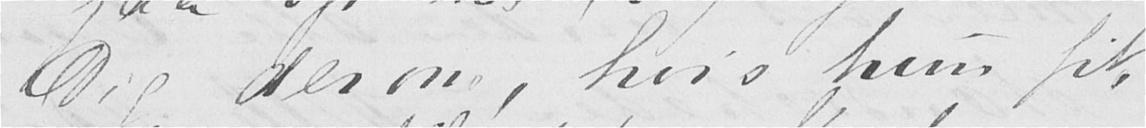Dig derom, hvis hun fik
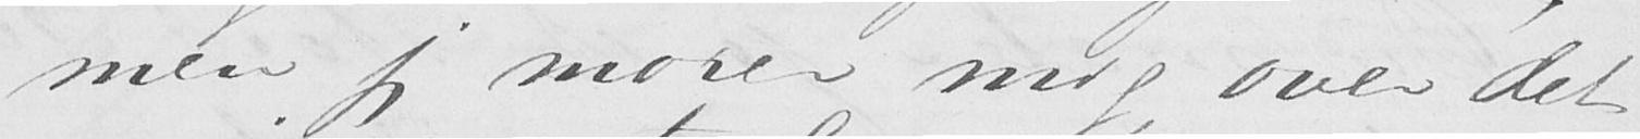men jeg morer mig over det
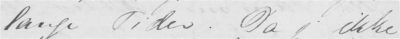lange Tider. Da jeg ikke
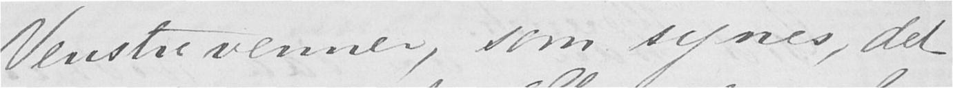Venstrevenner som synes, det
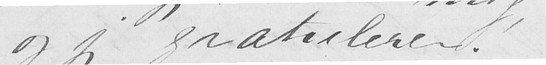og jeg gratulerer!
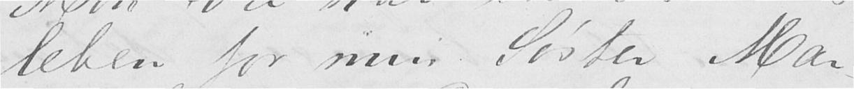leben for min Søster Mar-
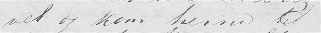vel og kom herned til
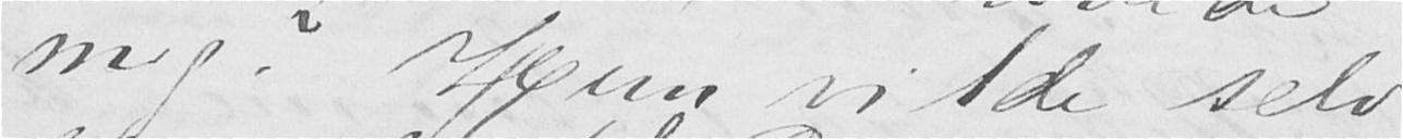mig? Hun vilde selv
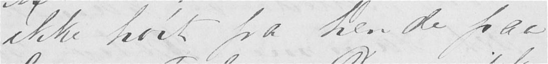ikke hørt fra hende paa
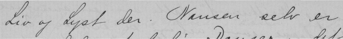Liv og Lyst der. Nansen selv er
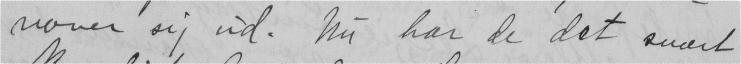vover sig ud. Nu har de det svært
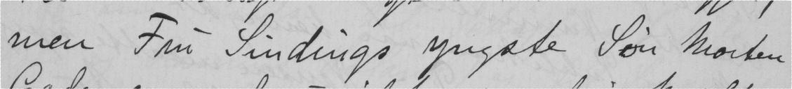men Fru Sindings yngste Søn Morten
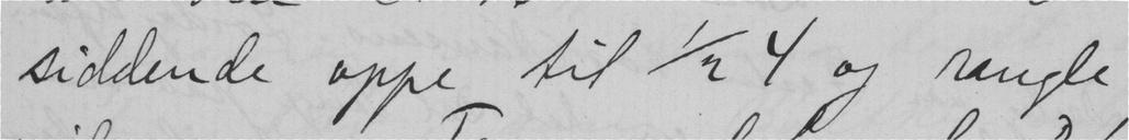siddende oppe til ½ 4 og rangle
